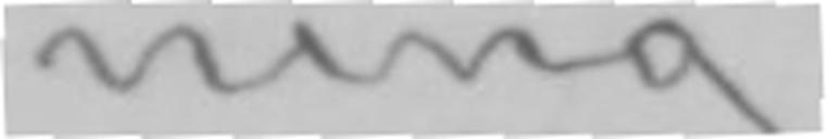ning?
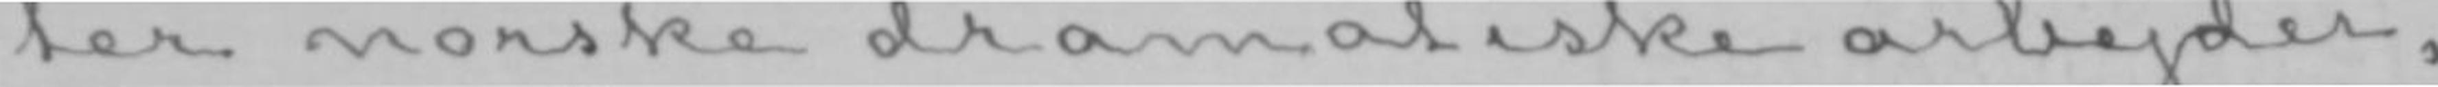ter norske dramatiske arbejder,
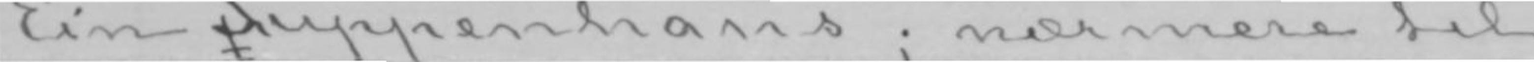«Ein pPuppenhaus»; nærmere til
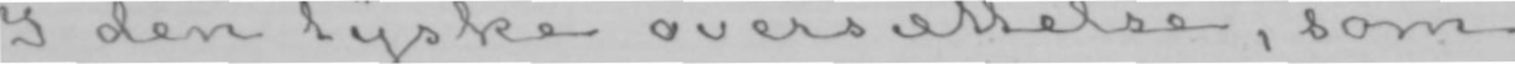I den tyske oversættelse, som
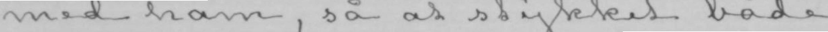med ham, så at stykket både
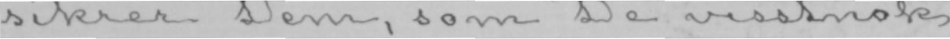sikrer dem, som De visstnok
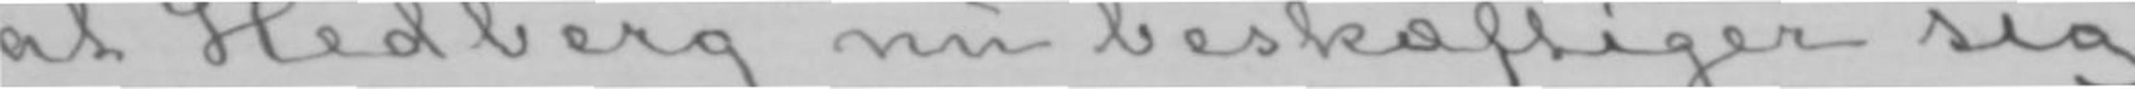at Hedberg nu beskæftiger sig
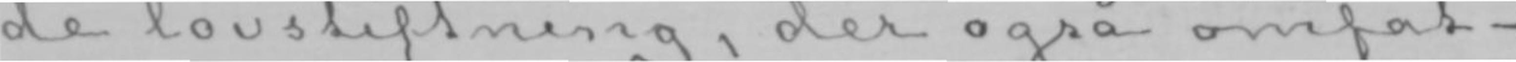de lovstiftning, der også omfat-
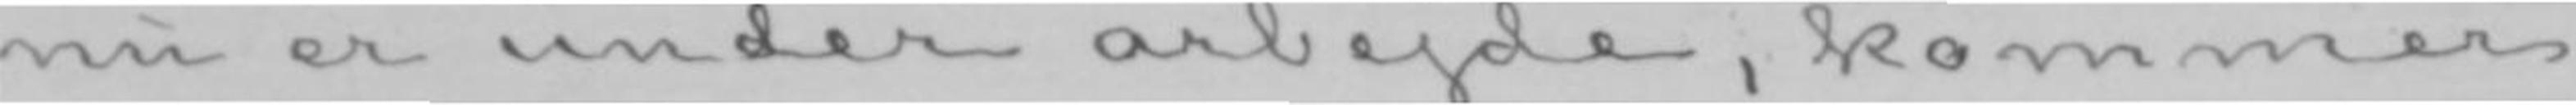nu er under arbejde, kommer
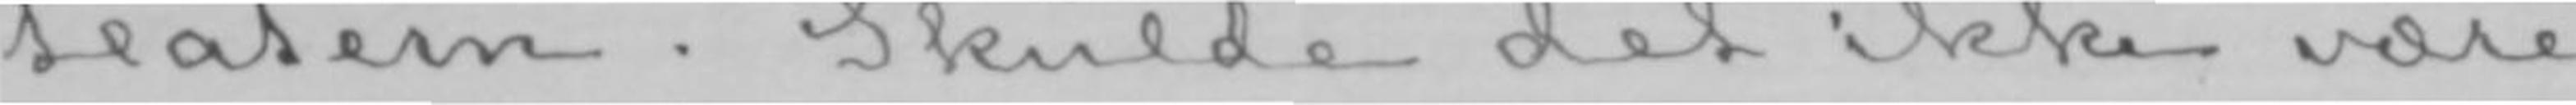teatern. Skulde det ikke være
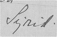Sigrid.
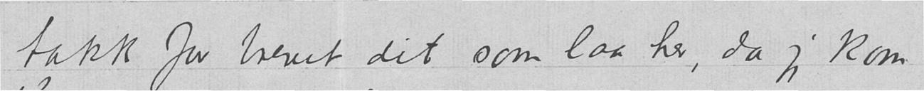takk for brevet dit som laa her, da jeg kom
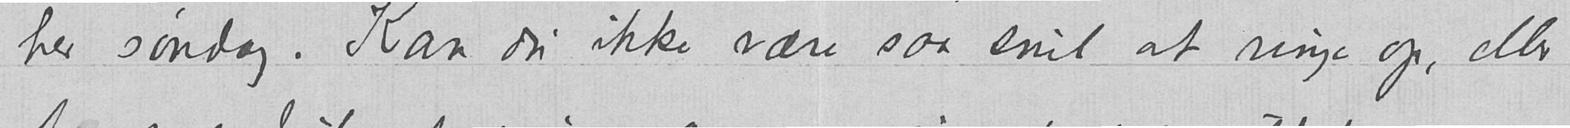her søndag. Kan du ikke være saa snil at ringe op, eller
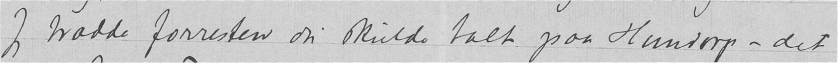Jeg trodde forresten du skulde talt paa Hundorp – det
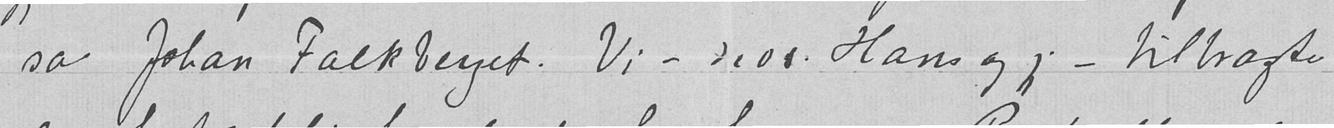sa Johan Falkberget. Vi – d.vs. Hans og jeg – tilbragte
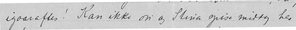igaaraftes! Kan ikke du og Stina spise middag her
